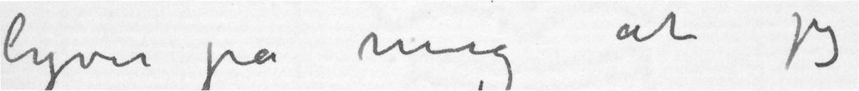lyver på mig at jeg
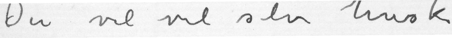Du vil vel selv huske
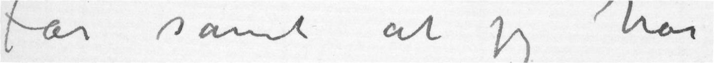Far samt at jeg har
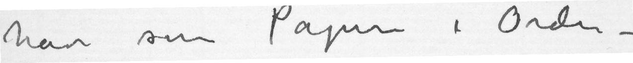have sine Papirer i Orden –
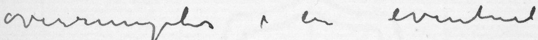overrumples i en eventuel
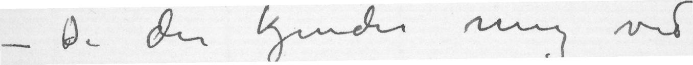– De der kjender mig ved
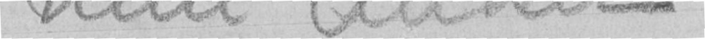Nini Anker
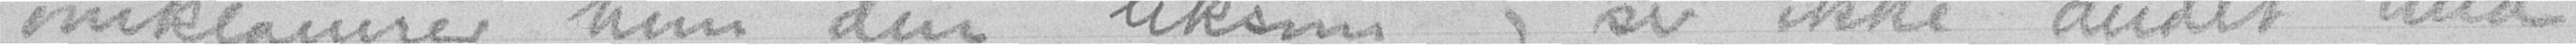omklamrer hun den liksom og ser ikke andet end
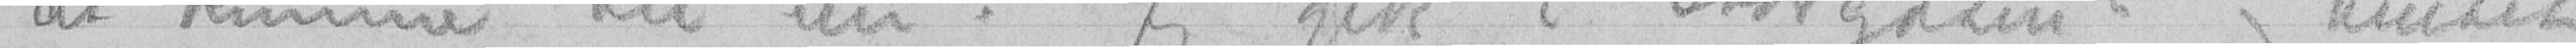at komme til nu. Jeg gik i "Storgaten" og ventet
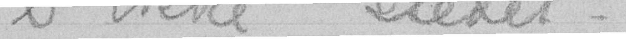er ikke stedet -
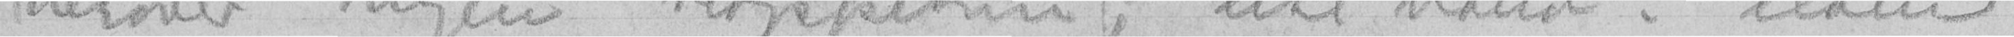nedover nogen trappetrin; lite vand i elven
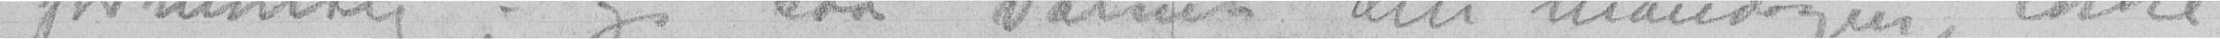formontlig; og saa varmt den mandagen, ikke
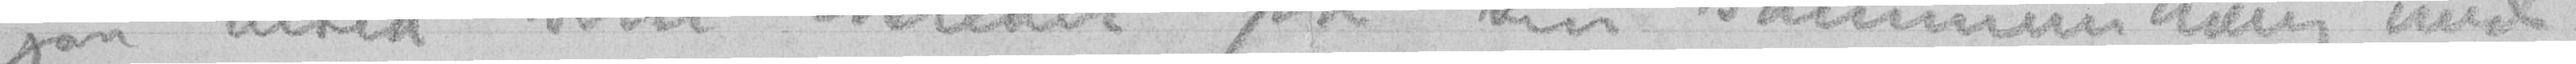ogsaa altid løsrevet fra sin sammengheng med
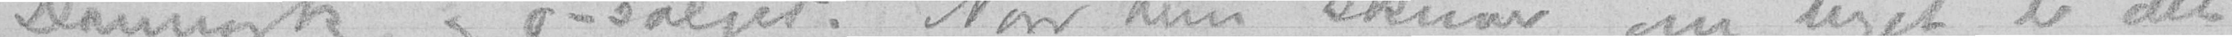Danmark og ø-salget. Naar hun skriver om noget er det
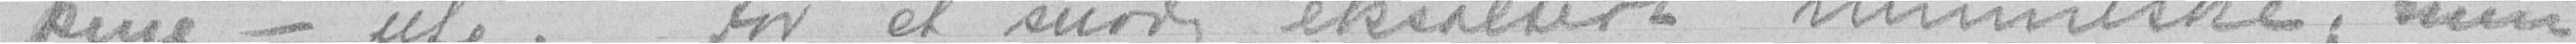pene - ute. For et snodig eksaltert menneske; men
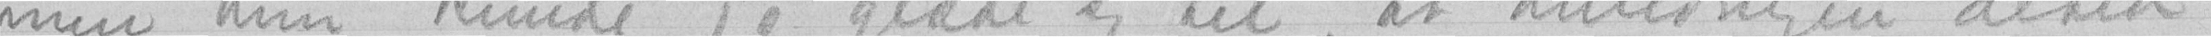men hun kunde jo glæde sig til, at anledningen altid
